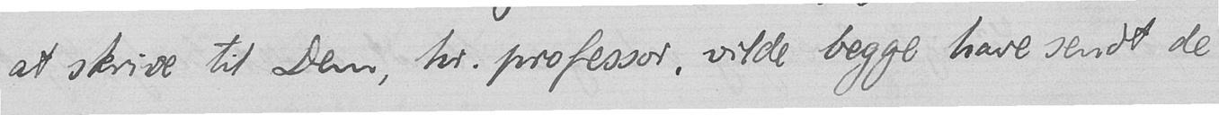at skrive til Dem, hr. professor, vilde begge have sendt de
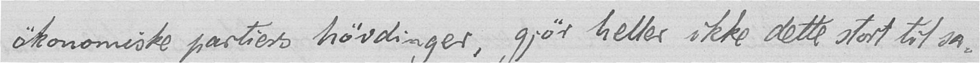økonomiske partiers høvdinger, gjør heller ikke dette stort til sa-
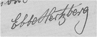Ebbe Hertzberg
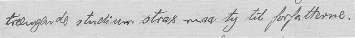trængende studium strax maa ty til forfatterne.
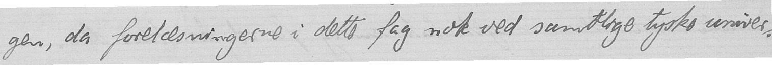gen, da forelæsningerne i dette fag nok ved samtlige tyske univer-
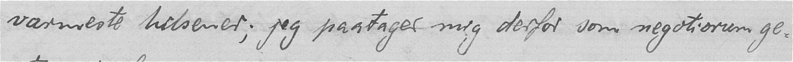varmeste hilsener; jeg paatager mig derfor som negotiorum ge-
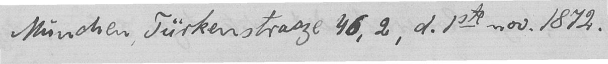München, Türkenstrasse 46, 2, d. 1ste nov. 1872.
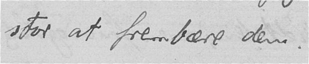stor at frembære dem.
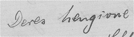Deres hengivne
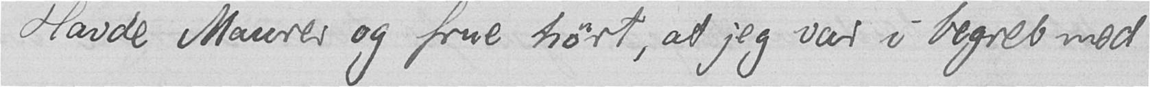Havde Maurer og frue hørt, at jeg var i begreb med
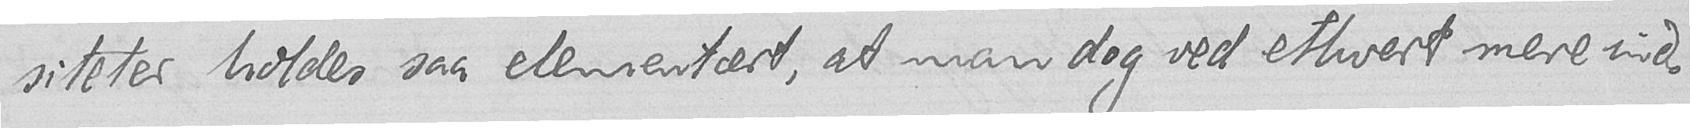siteter holdes saa elementært, at man dog ved ethvert mere ind-
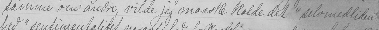samme om andre, vilde jeg maaske kalde det "selvmedliden-
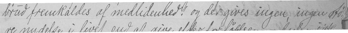brud fremkaldes af medlidenhed! og der gives ingen, ingen stør-
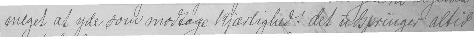meget at yde som modtage kjærlighed! det udspringer altid
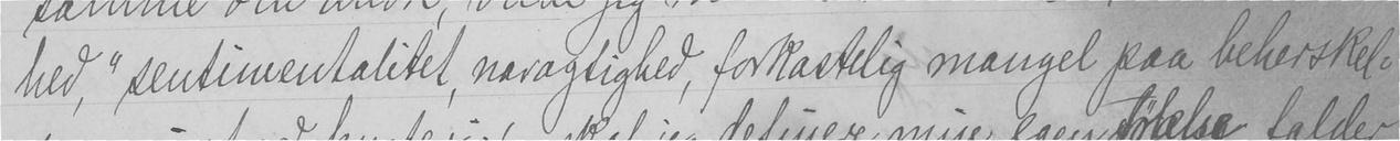hed," sentimentalitet, naraglighed, forkastelig mangel paa beherskel-
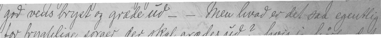god vens bryst og græde ud - - Men hvad er det saa egentlig
